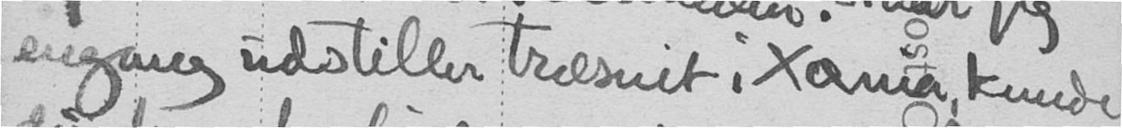engang udstiller træsnit Xania, kunde
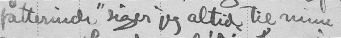fatterinde siger jeg altid til mine
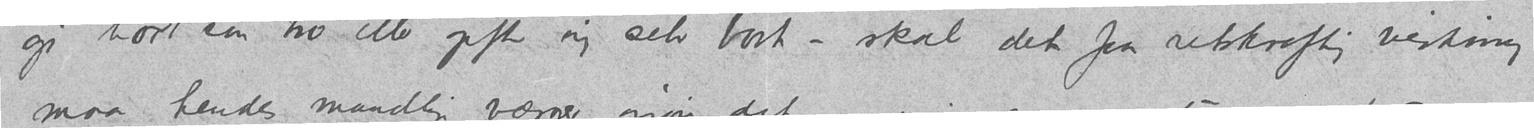gi bort sin tro eller gifte sig selv bort - skal det faa retskraftig virkning,
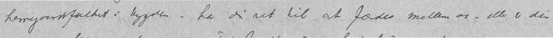herregaardfolket i bygden – har du ret til at færdes mellem os – eller er din
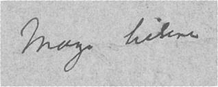Mange hilsener
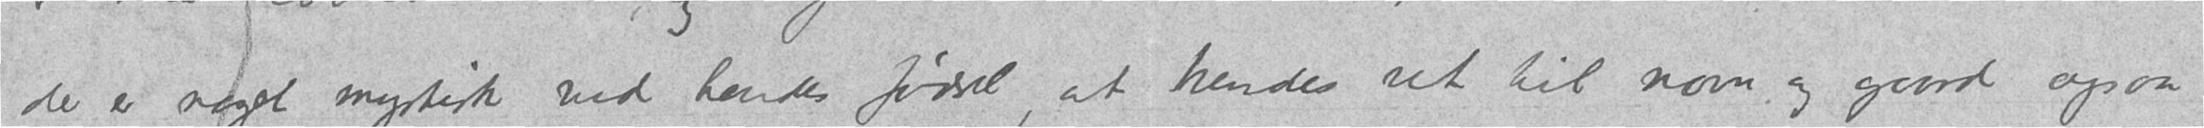der er noget mystisk ved hendes fødsel, at hendes ret til navn og gaard ogsaa
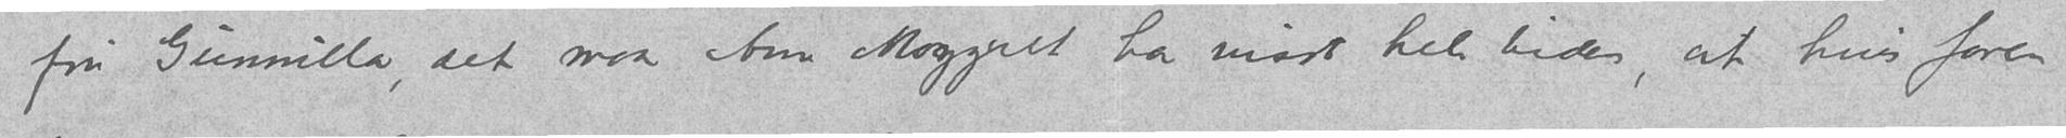fru Gunnilla, det maa Ann Maggret ha visst hele tiden, at hvis faren
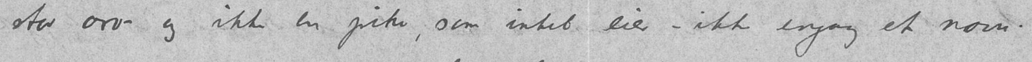stor arv - og ikke en pike, som intet eier – ikke engang et navn.
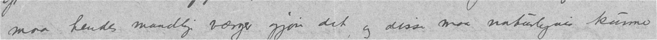maa hendes mandlige værger gjøre det, og disse maa naturligvis kunne
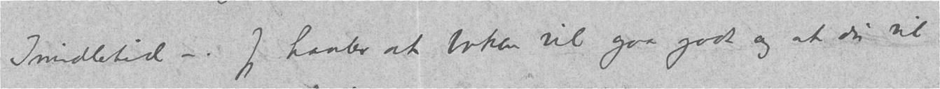Imidlertid –. Jeg haaber at boken vil gaa godt og at du vil
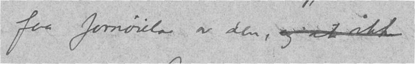faa fornøielse av den, og at ikke
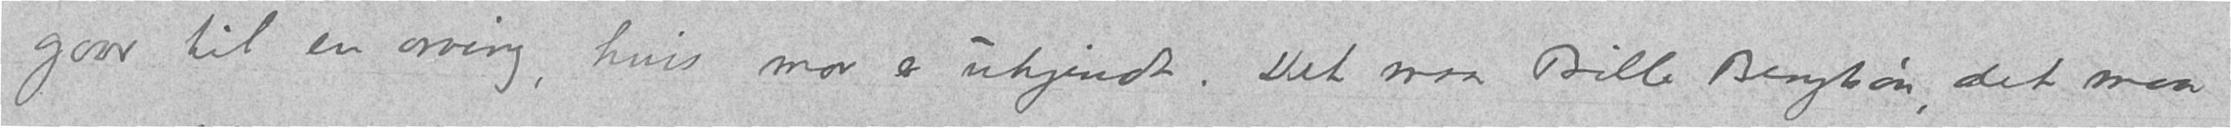gaar til en arving, hvis mor er ukjendt. Det maa Bille Bengtsøn, det maa
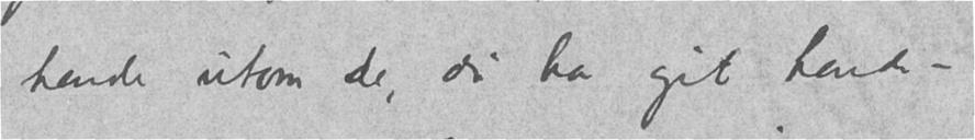hende utom de, du har git hende –.
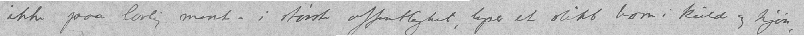ikke paa lovlig maate – i største offentlighet, lyser et slikt barn i kulde og kjøn,
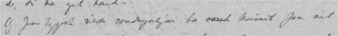Og Jens Eggert vilde sandsynligvis ha været kunnet faa sit
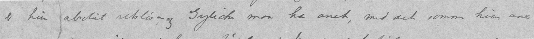er hun absolut retsløs – og Gylicke maa ha anet, med det samme hun aner
